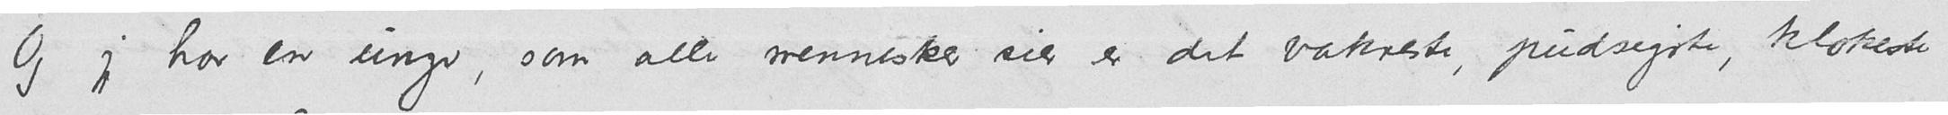Og jeg har en unge, som alle mennesker sier er det vakreste, pudsigste, klokeste
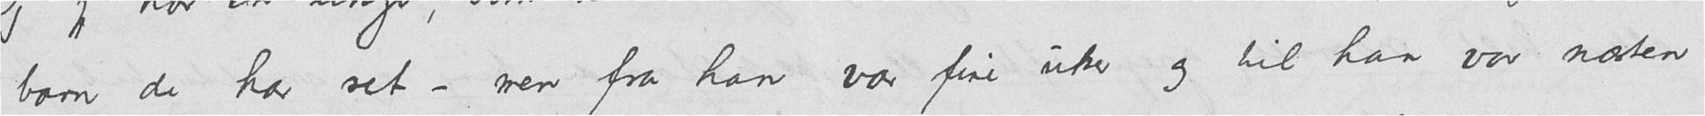barn de har set - men fra han var fire uker og til han var næsten
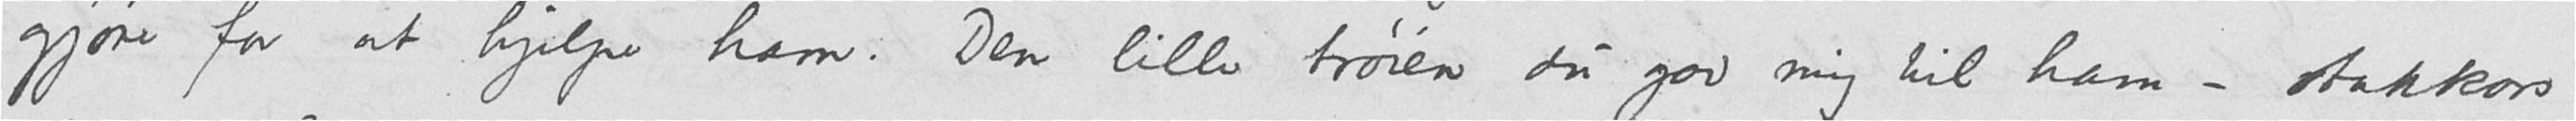gjøre for at hjelpe ham. Den lille trøien du gav mig til ham - stakkars
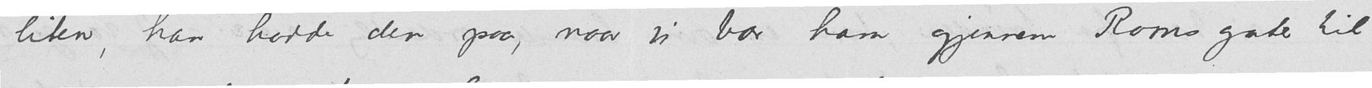liten, han hadde den paa, naar vi bar ham gjennem Roms gater til
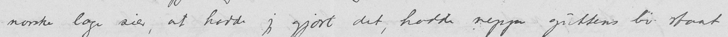norske læge sier, at hadde jeg gjort det, hadde neppe guttens liv staat
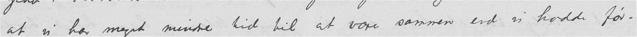at vi har meget mindre tid til at være sammen end vi hadde før.
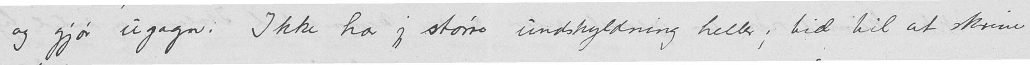og gjør ugagn: Ikke har jeg større undskyldning heller; tid til at skrive
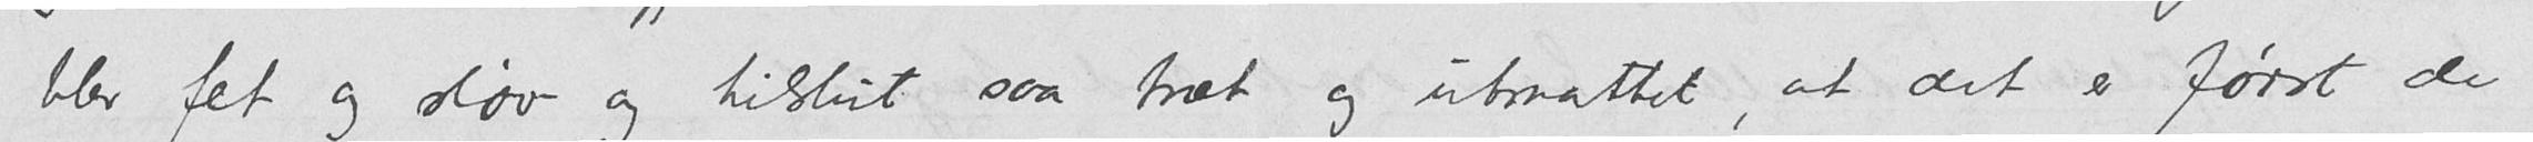blev fet og sløv og tilslut saa træt og utmattet, at det er først de
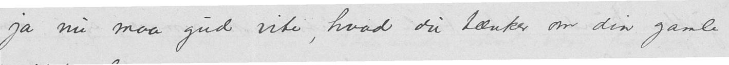ja nu maa gud vite, hvad du tænker om din gamle
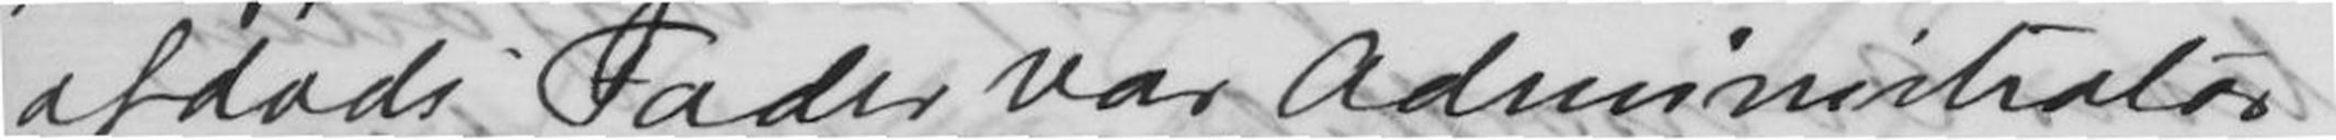afdøde Fader var Administrator
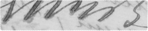Ellen B.
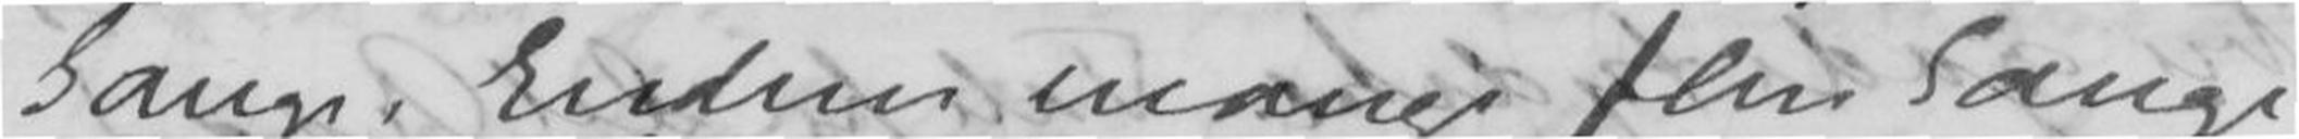Gange. Endnu mange flere Gange
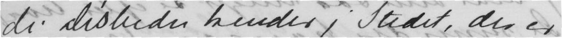de. Desbedre kender j Stedet, der er
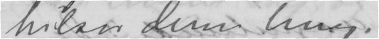hilser Dem heng.
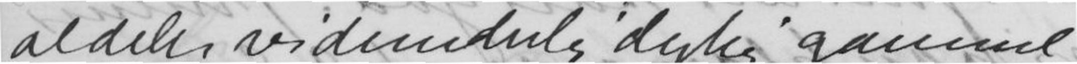aldeles vidunderlig dejlig gammel
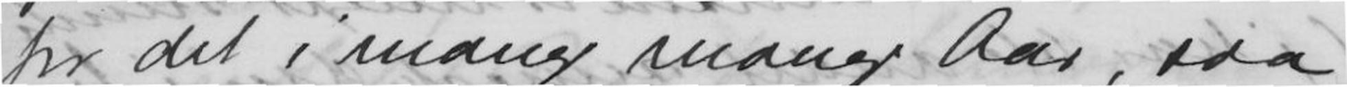for det i mange mange Aar, saa
